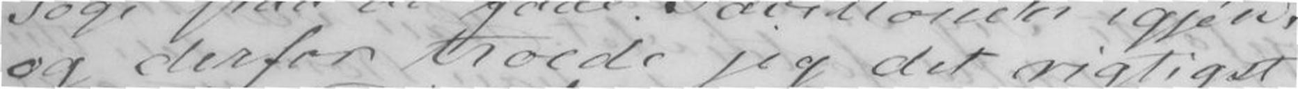og derfor troede jeg det rigtigst
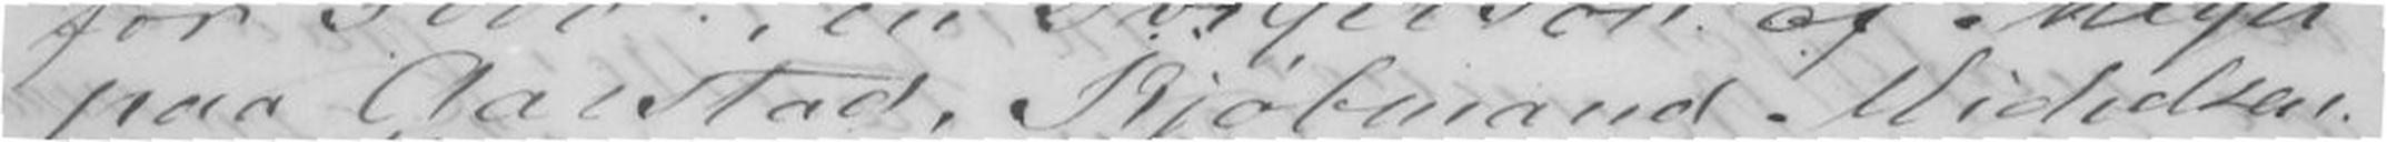paa Aarstad, Kjøbmand Michelsen
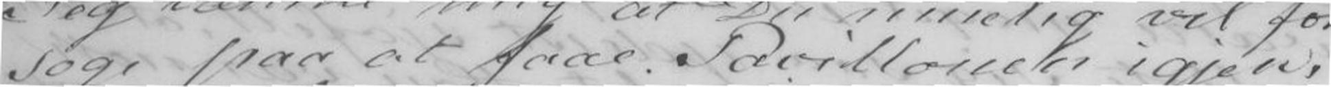søge paa at faae Pavillonen igjen,
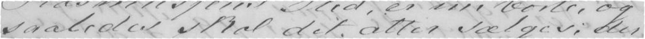saaledes skal det atter sælges, der
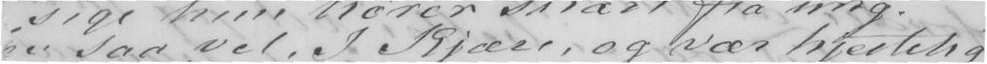saa vel, I kjære, og vær hjertelig
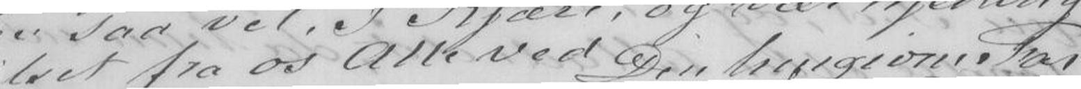lset fra os Alle ved Din hengivne Far
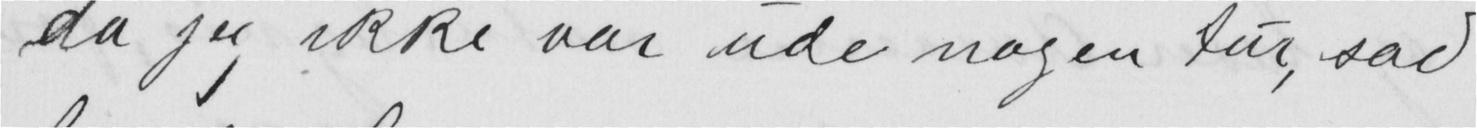da jeg ikke var ude nogen tur, sad
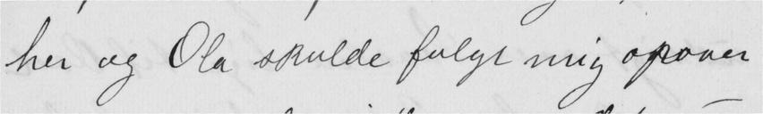her og Ola skulde følge mig opover
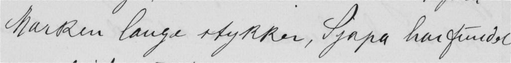Marken lange stykker, Sjapa har fundet
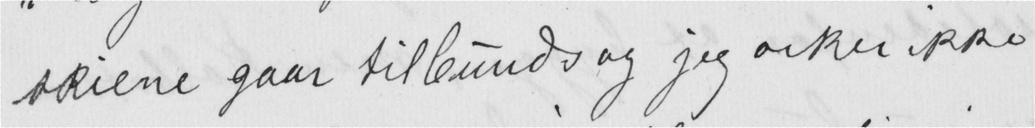skiene gaar tilbunds og jeg orker ikke
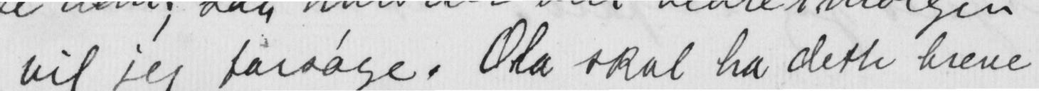til jeg forsøge. Ola skal ha dette breve
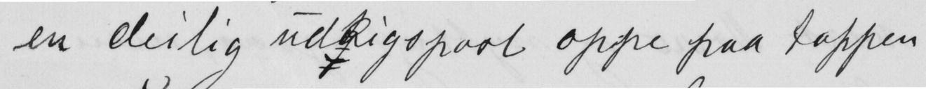en deilig udkigspost oppe paa Toppen
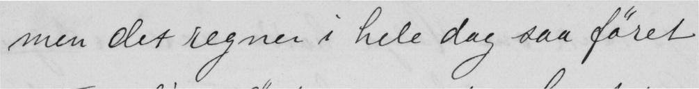men det regner i hele dag saa føret
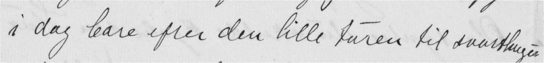i dag bare efter den lille turen til svartlingen
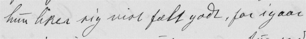hun liker sig mot fælt godt, for igaar
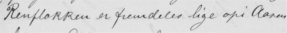Renflokken er fremdeles lige opi Aaren
